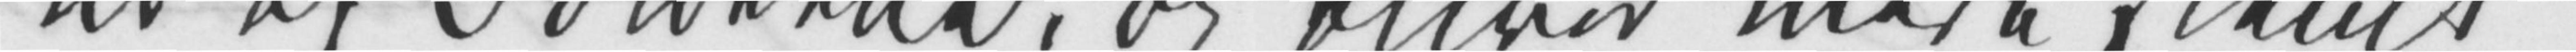ud af Folderne, og bliver mere stemt
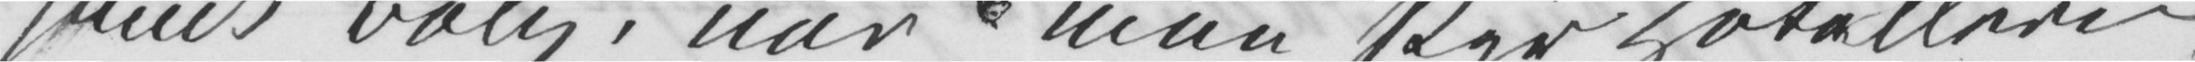smuk Bolig, når man skyr Hotellerne
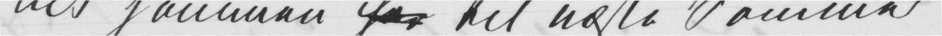lidt sammen her til næste Sommer
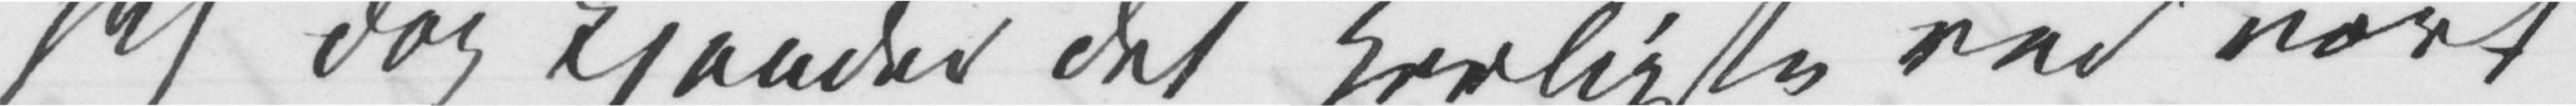jeg dog kjender det Herligste ved vort
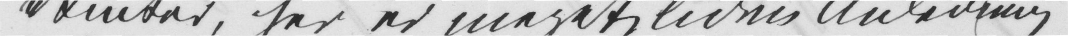Vinter, her er meget liden Anledning
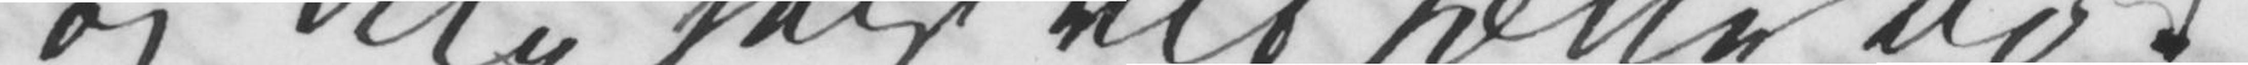og ikke selv vil sætte Bo.
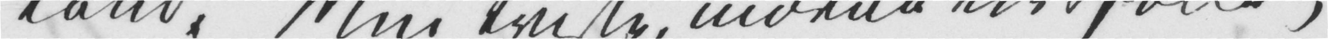Land! Min triste, moene Livsfælle,
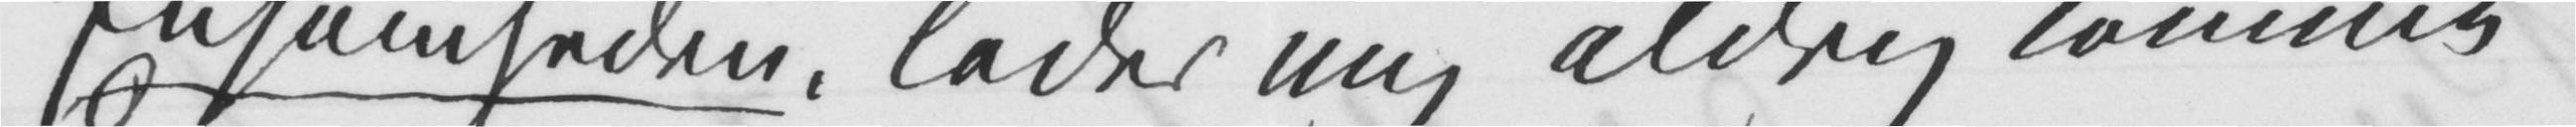Ensomheden, lader mig aldrig komme
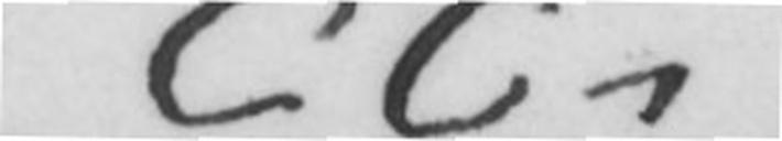CC.
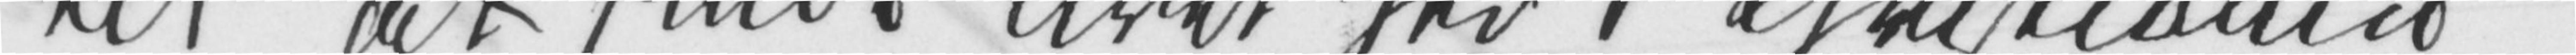til at finde Livet her i Christiania
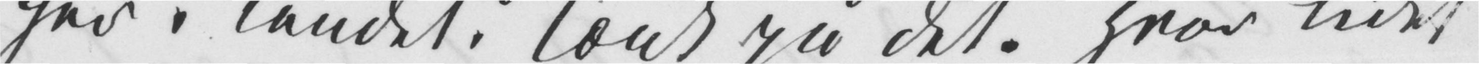her i Landet? Tænk på det. Hvor lidet
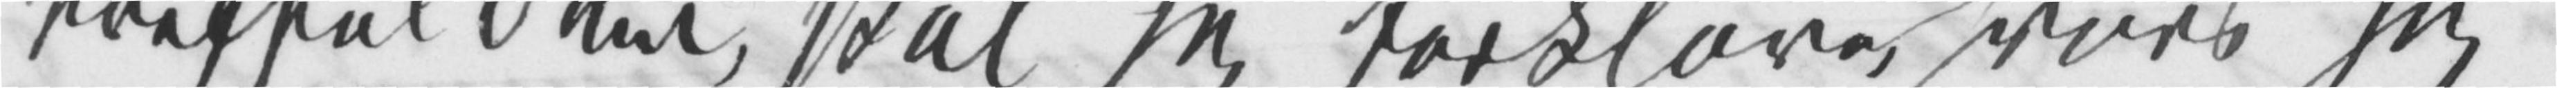treffe Dem, skal jeg forklare, hvori jeg
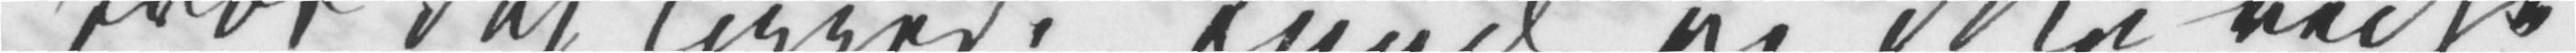tror det ligger. Kunde vi ikke reise
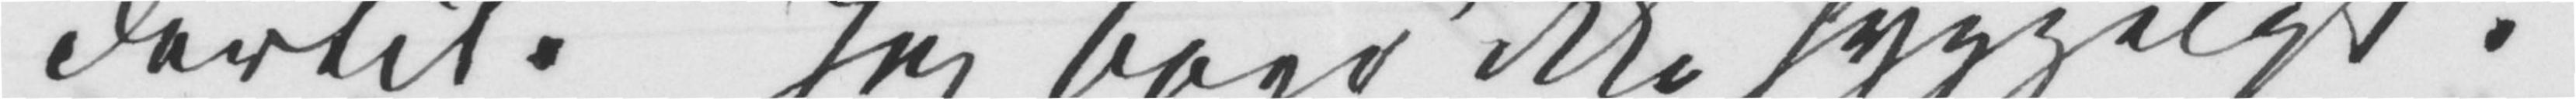dertil. Jeg boer ikke hyggeligt i
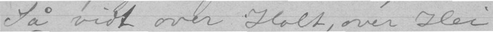Så vidt over Holt, over Hei
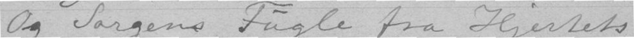Og Sorgens Fugle fra Hjertets
